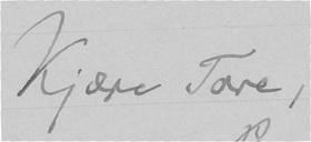Kjære Tore,
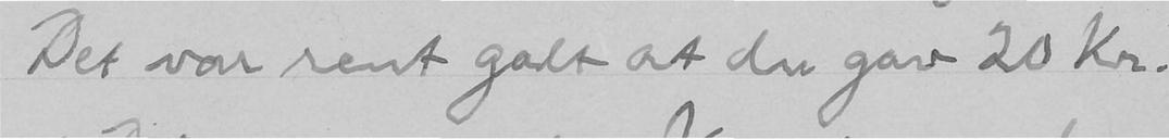Det var rent galt at du gav 20 Kr.
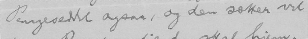Pengeseddel ogsaa, og den sætter vel
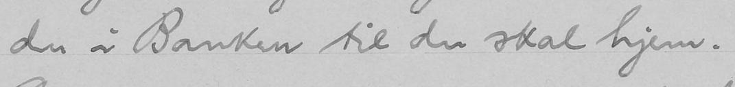du i Banken til du skal hjem.
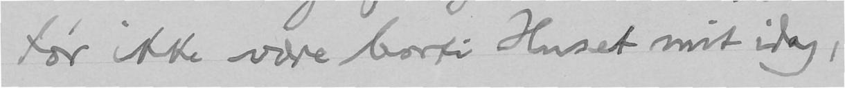tør ikke være borti Huset mit idag,
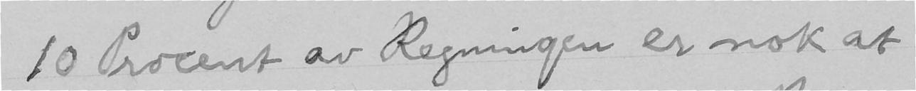10 Procent av Regningen er nok at
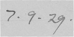7.9.29.
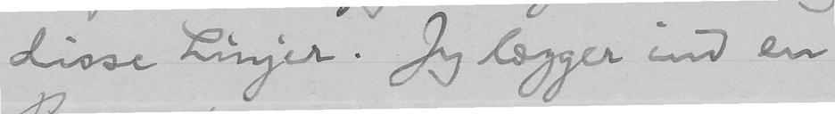disse Linjer. Jeg lægger ind en
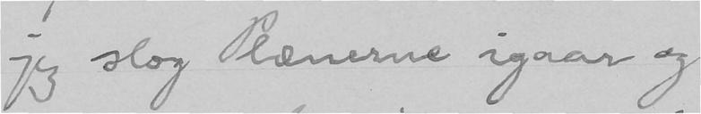jeg slog Plænerne igaar og
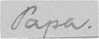Papa.
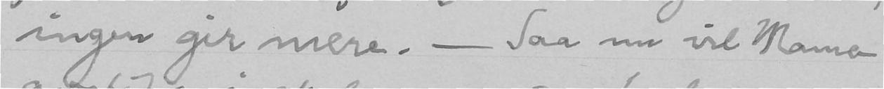ingen gir mere. - Saa nu vil Mama
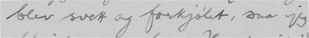blev svett og forkjølet, saa jeg
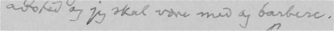avsted og jeg skal være med og barbere.
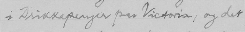i Drikkepenger paa Victoria, og det
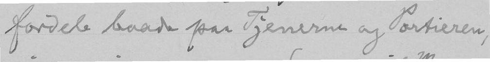fordele baade paa Tjenerne og Portieren,
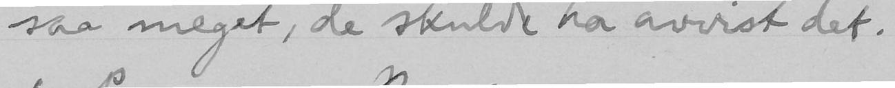saa meget, de skulde ha avvist det.
